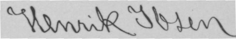Henrik Ibsen.
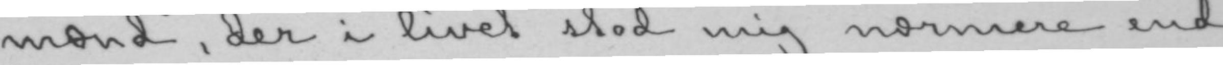mænd, der i livet stod mig nærmere end
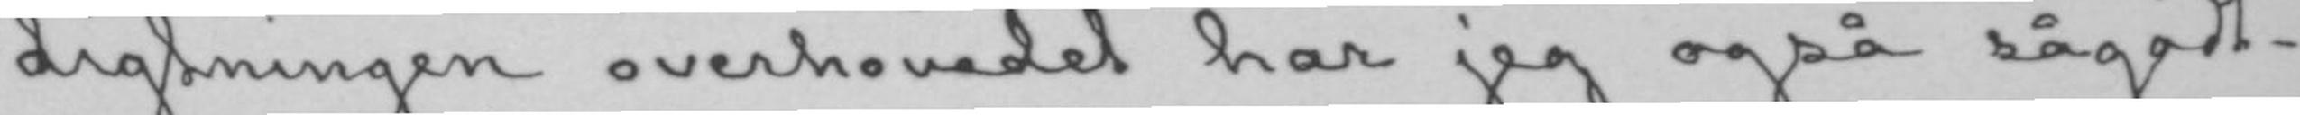digtningen overhovedet har jeg også sågodt-
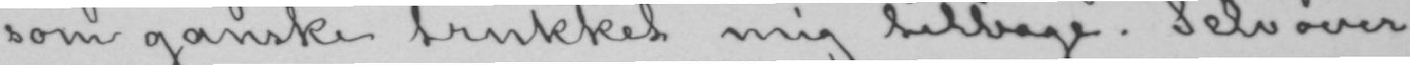som ganske trukket mig tilbage. Selv over
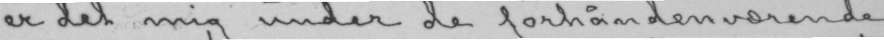er det mig under de forhåndenværende
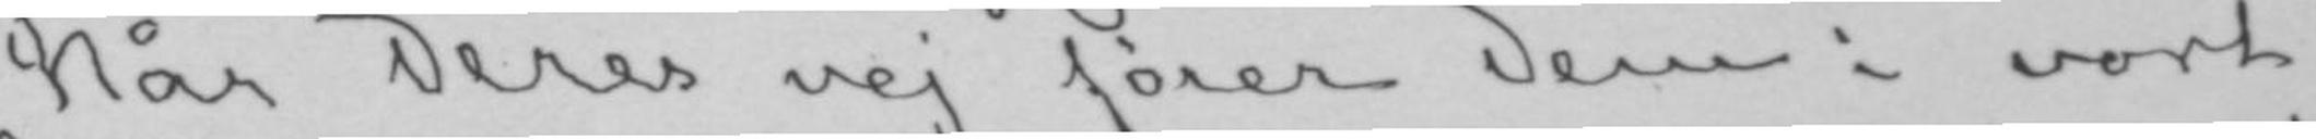Når Deres vej fører Dem i vort
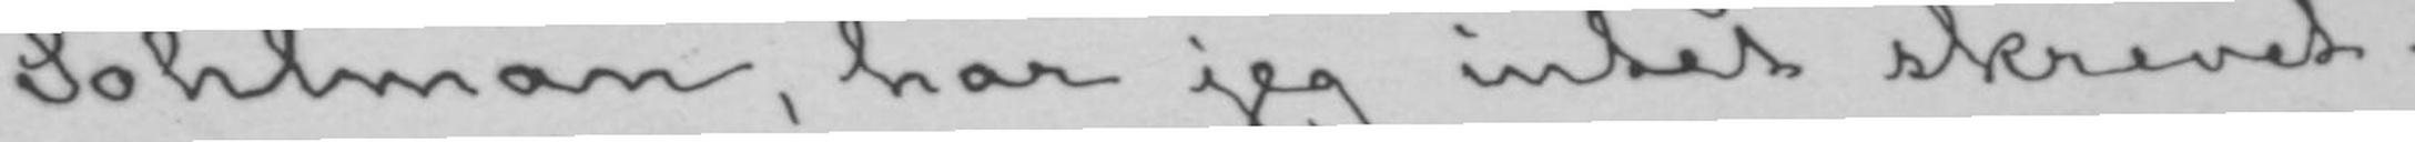Sohlman, har jeg intet skrevet.
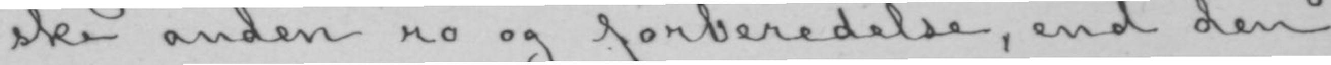ske anden ro og forberedelse, end den,
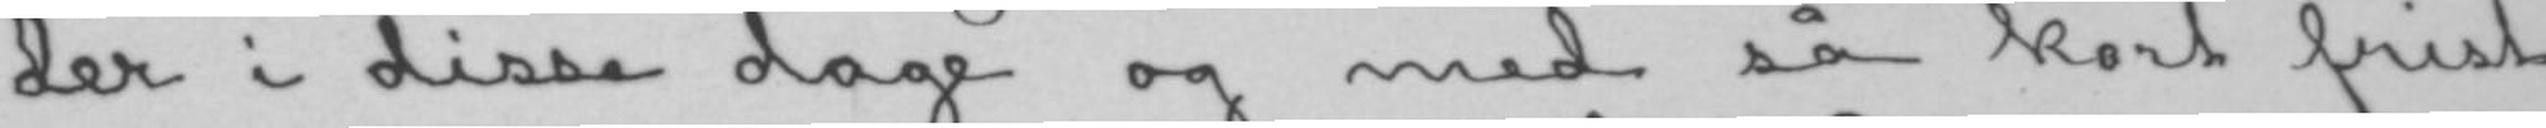der i disse dage og med så kort frist
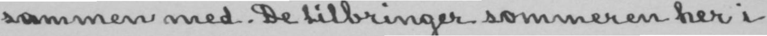sammen med. De tilbringer sommeren her i
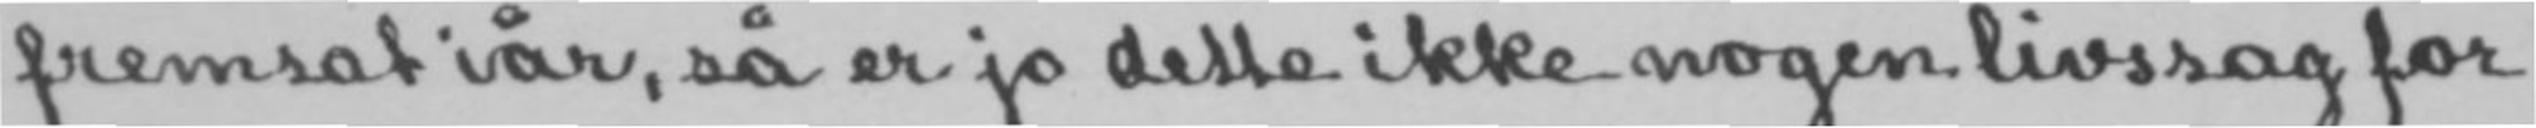fremsat iår, så er jo dette ikke nogen livssag for
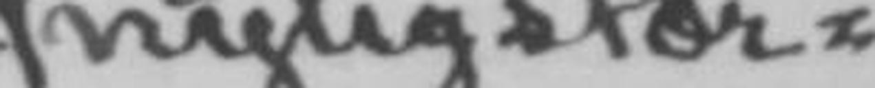nylig stor-
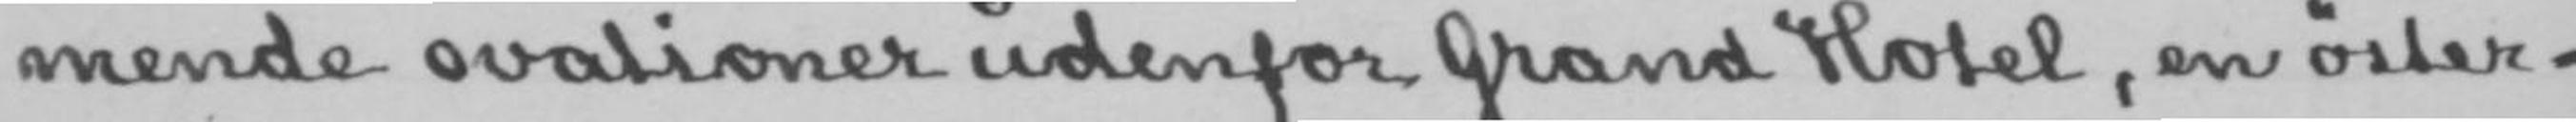mende ovationer udenfor Grand Hotel, en øster-
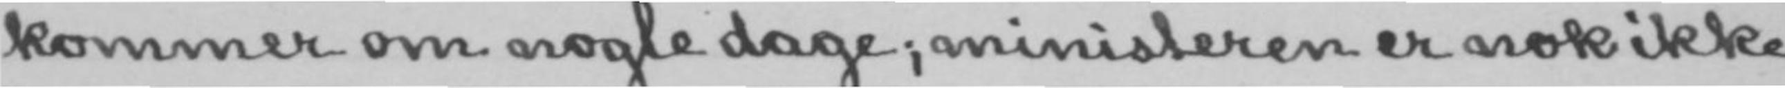kommer om nogle dage; ministeren er nok ikke
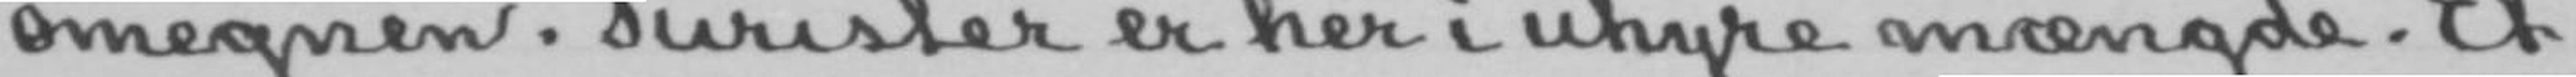omegnen. Turister er her i uhyre mængde. Et
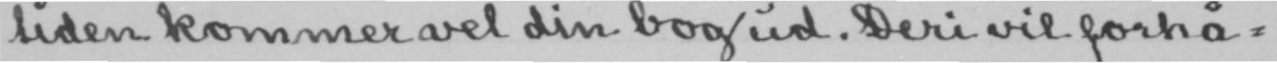tiden kommer vel din bog ud. Deri vil forhå-
